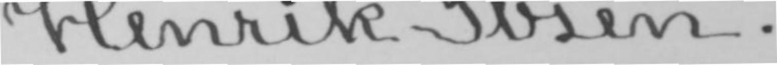Henrik Ibsen.
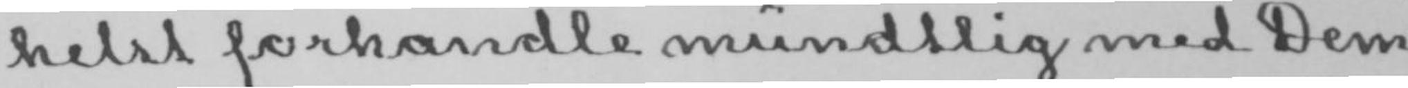helst forhandle mundtlig med Dem
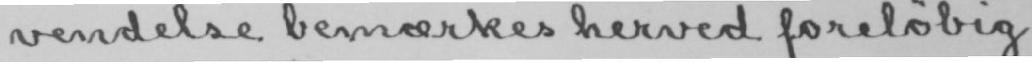vendelse bemærkes herved foreløbig
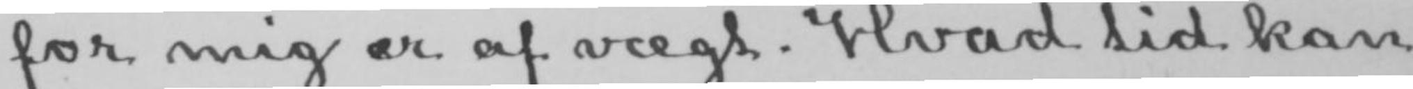for mig er af vægt. Hvad tid kan
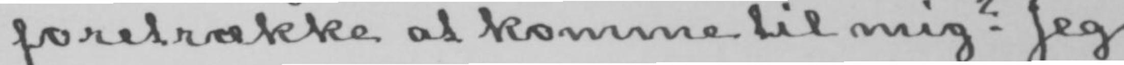foretrække at komme til mig? Jeg
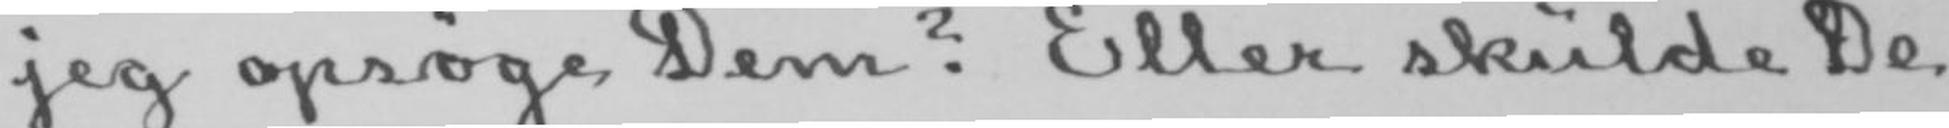jeg opsøge Dem? Eller skulde De
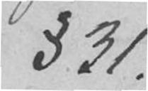§ 31.
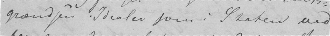grændsen. Idealer som Staten ved
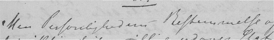Men Personlighedens Bestemmelse og
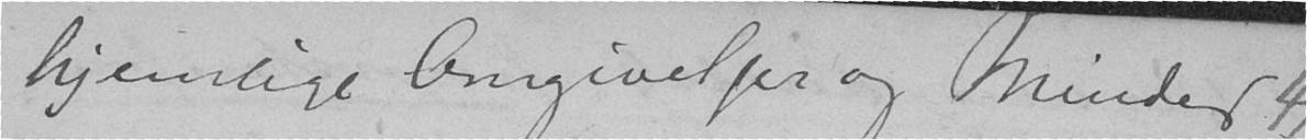hjemlige Omgivelser og Minder
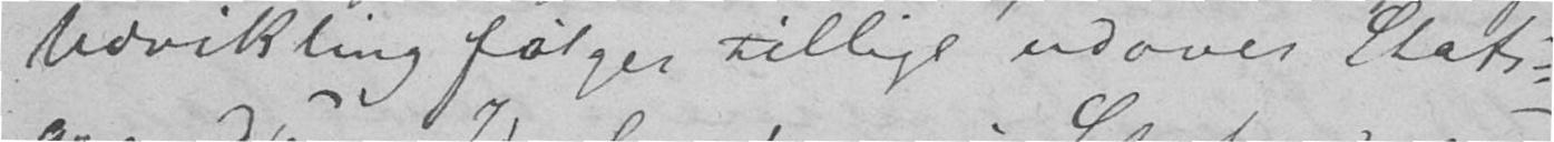Udvikling følger tillige udover Stats-
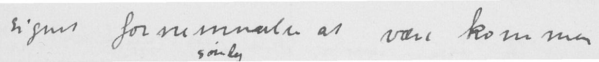signet fornemmelse at være kommen
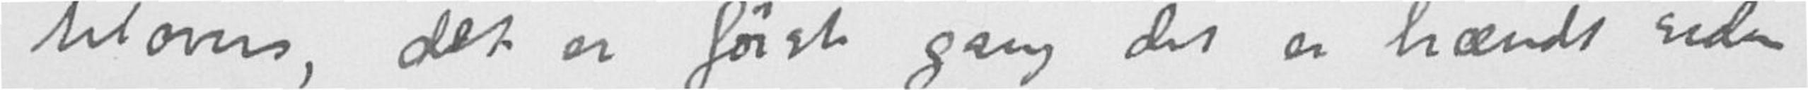tilovers, det er første gang det er hændt siden
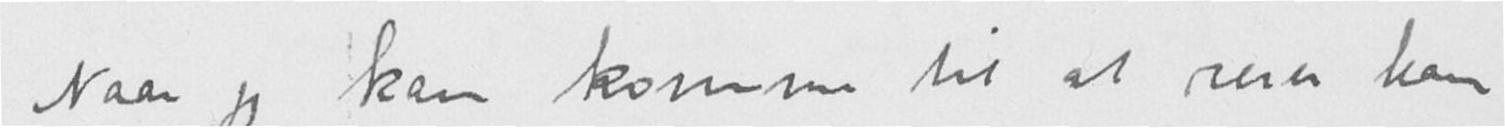Naar jeg kan komme til at reise kan
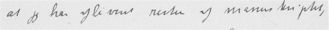at jeg har afleveret resten af manuskriptet,
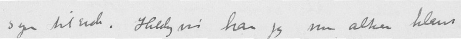syn tilside. Heldigvis har jeg nu altsaa klart
